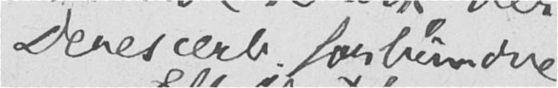Deres ærb. forbundne
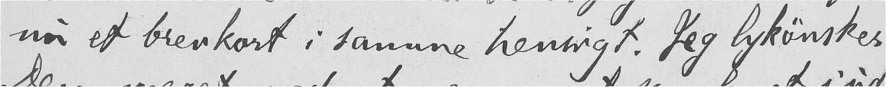nu et brevkort i samme hensigt. Jeg lykønsker
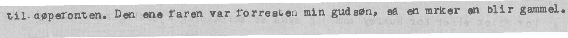til døpefonten. Den ene faren var forresten min gudsøn, så en mrker en blir gammel.
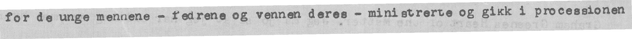for de unge mennene - fedrene og vennen deres - ministrerte og gikk i processionen
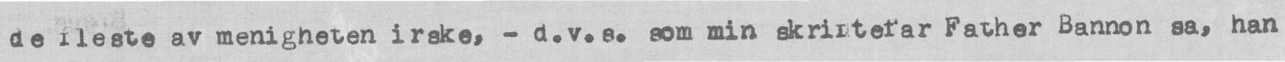de fleste av menigheten irske, - d.v.s. som min skrirtefar Father Bannon sa, han
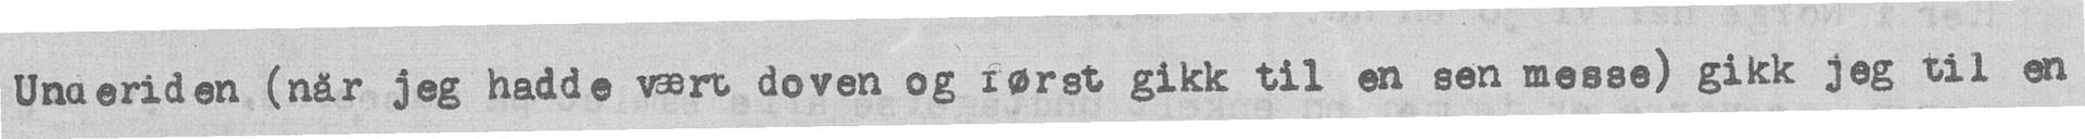Underiden (när jeg hadde vært doven og først gikk til en sen messe) gikk jeg til en
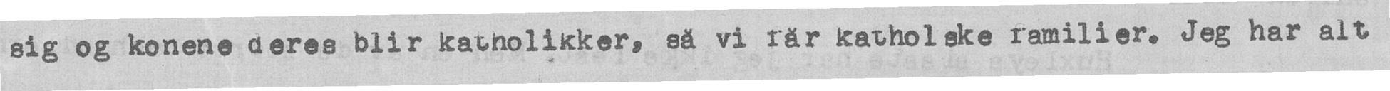sig og konene deres blir katholikker, så vi fär katholske familier. Jeg har alt
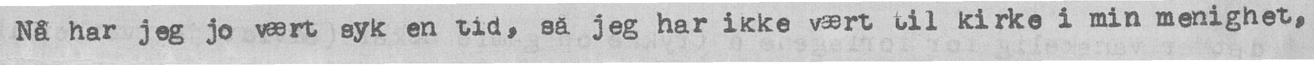Nå har jeg jo vært syk en tid, så jeg har ikke vært til kirke i min menighet,
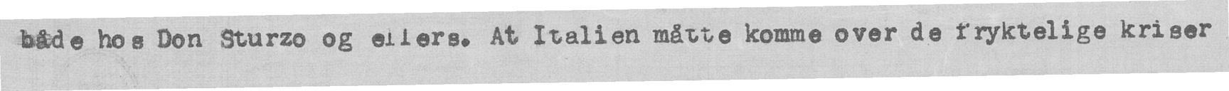både hos Don Sturzo og ellers. At Italien måtte komme over de fryktelige kriser
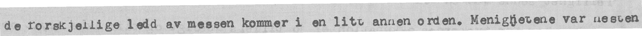de forskjellige ledd av messen kommer i en litt annen orden. Menigjebene var nesten
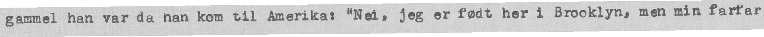gammel han var da han kom til Amerika: „Nei, jeg er født her i Brooklyn, men min farfar
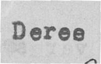Deres
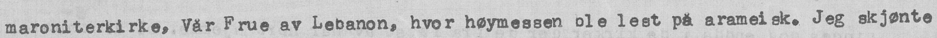maroniterkirke, Vår Frue av Lebanon, hvor høymessen ole lest på arameisk. Jeg skjønte
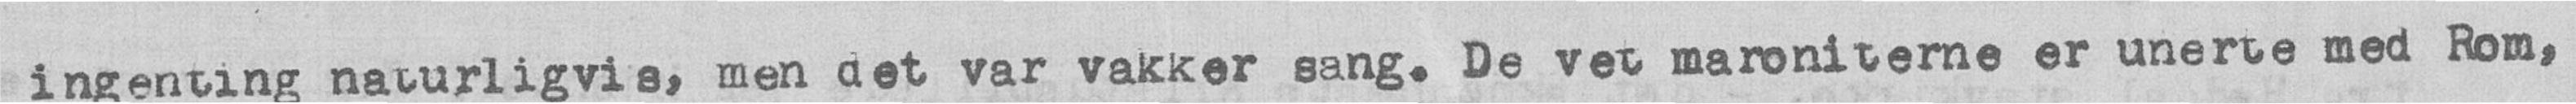ingenting naturligvis, men det var vakker sang. De vet maroniterne er unerte med Rom,
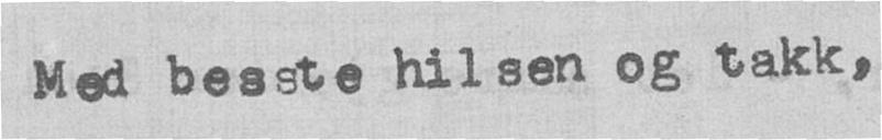Med besste hilsen og takk,
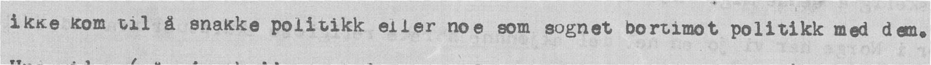ikke kom til å snakke politikk eller noe som sognet bortimot politikk med dem.
